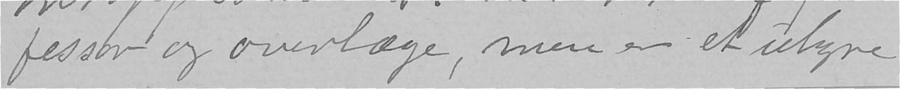fessor og overlæge, men er et uhyre
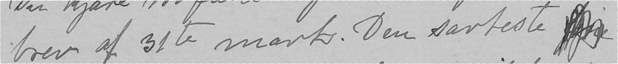brev af 31te marts. Den sarteste fine
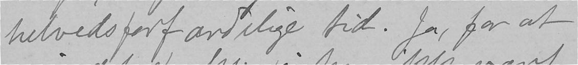helvedsforfærdelige tid. Ja, for at
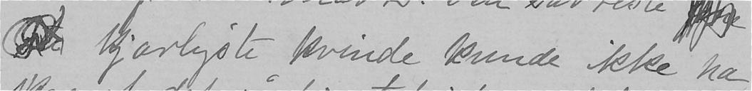De kjærligste kvinde kunde ikke ha
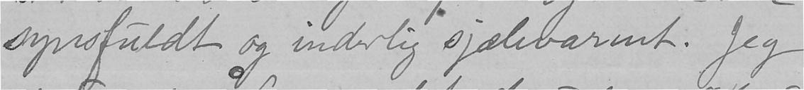synsfuldt og inderlig sjælevarmt. Jeg
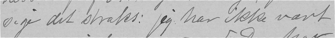sige det straks: jeg har ikke vært
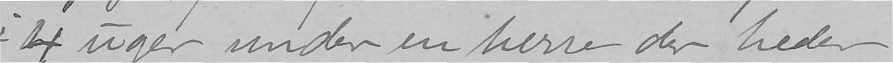i 4 uger under en herre der heder
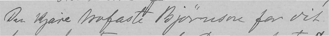Du kjære trofaste Bjørnson for dit
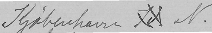Kjøbenhavn N.
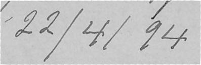22/4/94
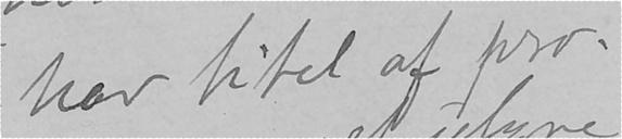har titel af pro-
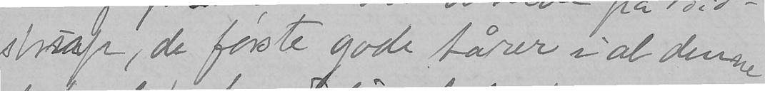strup, de første gode tårer i al denne
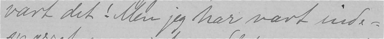vært det! Men jeg har vært inde-
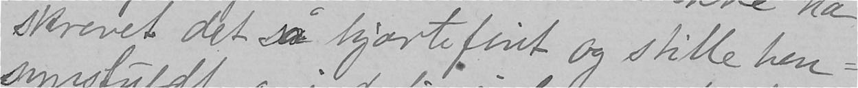skrevet det sa hjærtefint og stille hen-
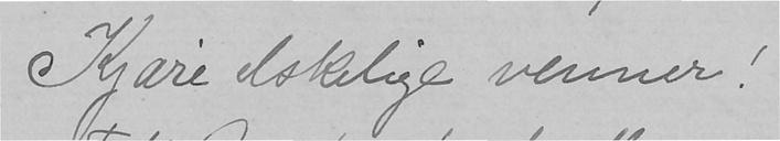Kjære elskelige venner!
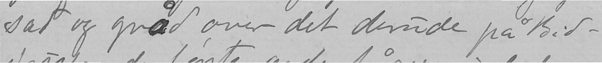sad og gråd over det derude på Bid-
